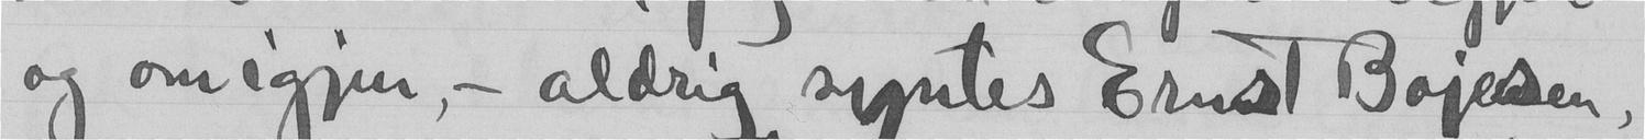og omigjen, - aldrig syntes Ernst Bojesen,
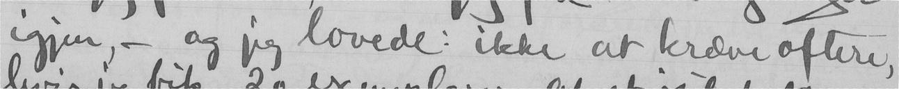igjen, - og jeg lovede: ikke at kræve oftere,
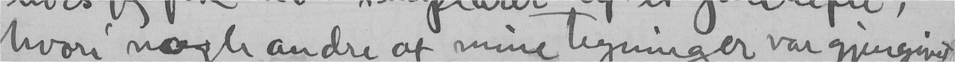hvori nogle andre af mine tegninger var gjengivet
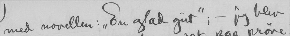med novellen: "En glad gut" ; - jeg blev
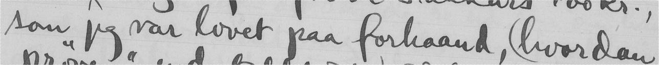som jeg var lovet paa forhaand, (hvordan
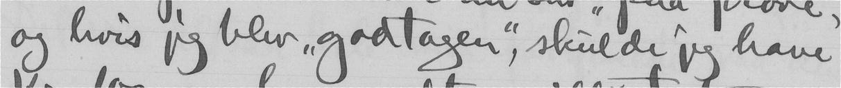og hvis jeg blev "godtagen" skulde jeg have
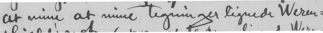at mine at mine tegninger lignede Weren-
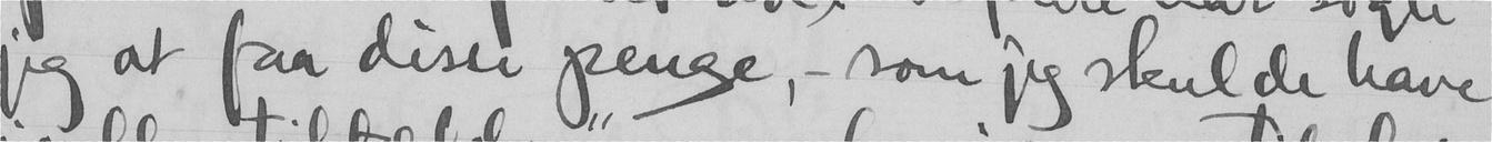jeg at faa disse penge, - som jeg skulde have
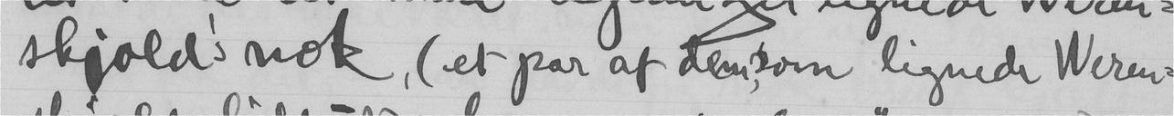skjold's nok, (et par af den, som lignede Weren-
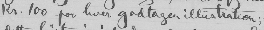Kr. 100 for hver godtagen illustration;
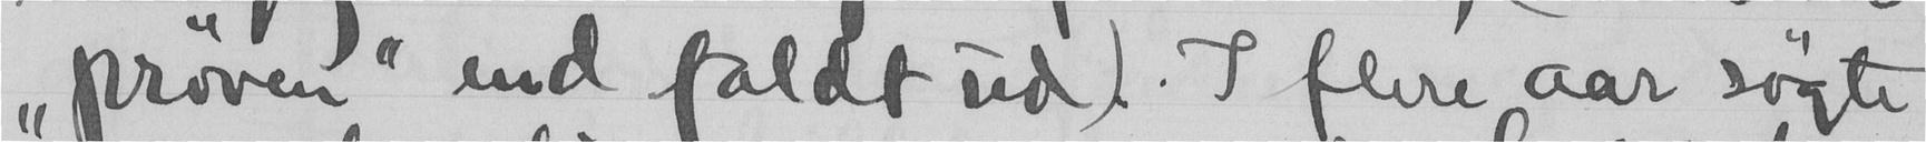"prøven" end faldt ud). I flere aar søgte
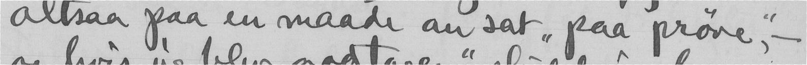altsaa paa en maade ansat "paa prøve", -
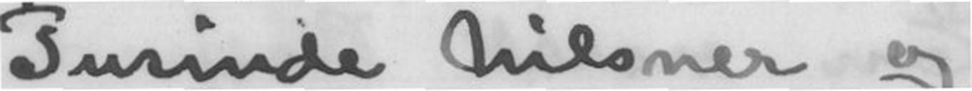Tusinde hilsner og
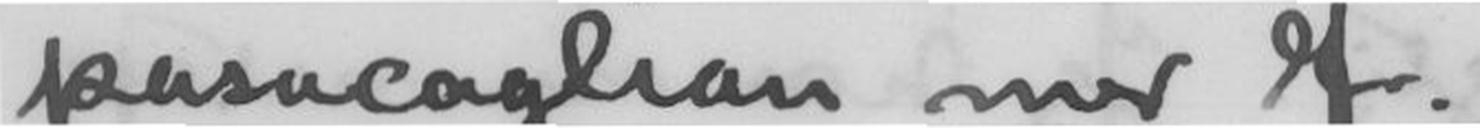pasacaglian med Hr.
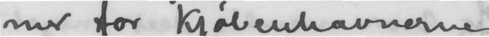med for Kjøbenhavnerne
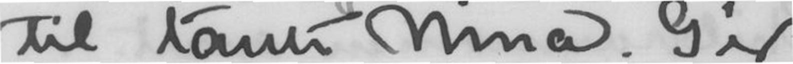til tante Nina. Gid
